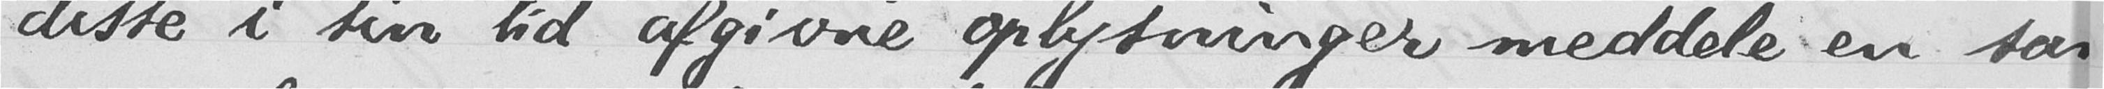disse i sin tid afgivne oplysninger meddele en sam-
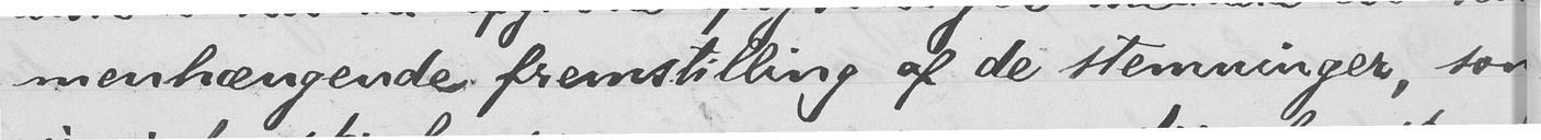menhængende fremstilling af de stemninger, som
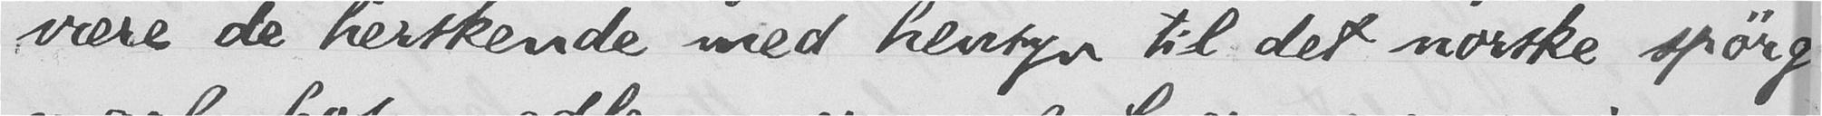være de herskende med hensyn til det norske spørgs-
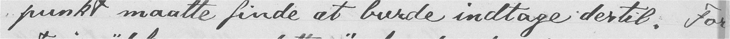punkt maatte finde at burde indtage dertil. For
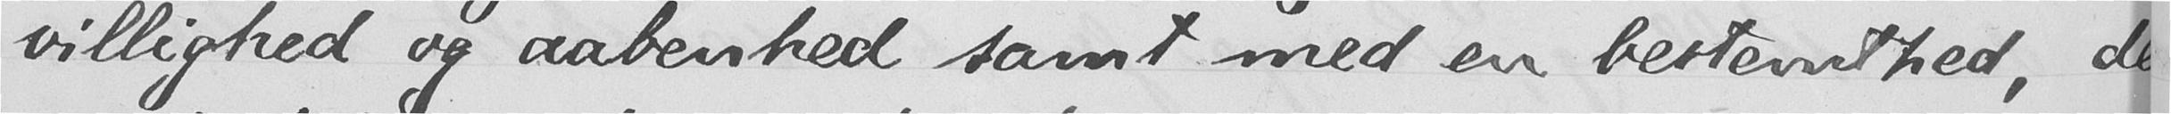villighed og aabenhed samt med en bestemthed, der
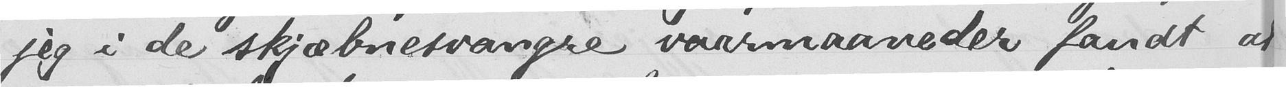jeg i de skjæbnesvangre vaarmaaneder fandt at
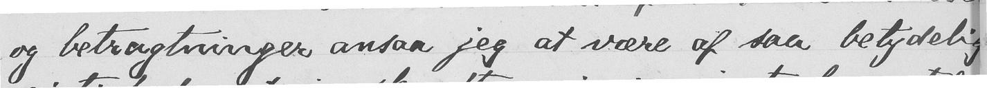og betragtninger ansaa jeg at være af saa betydelig
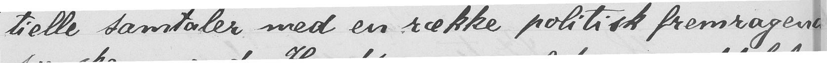tielle samtaler med en række politisk fremragende
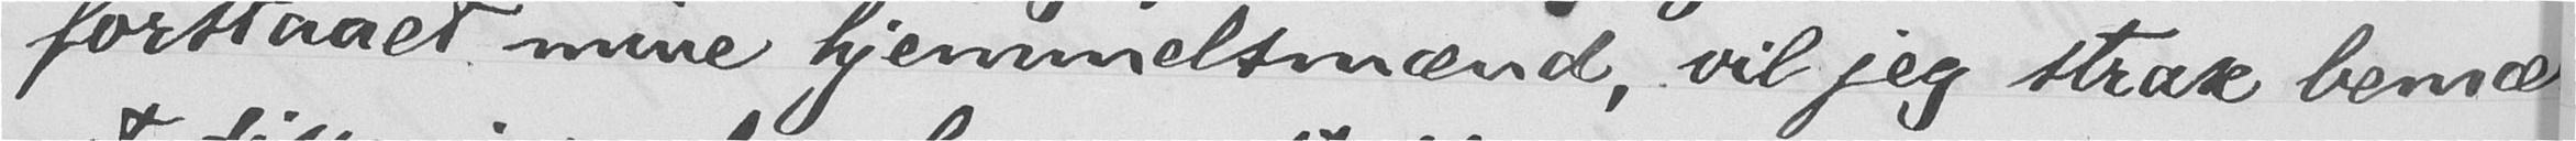forstaaet mine hjemmelsmænd, vil jeg strax bemærke
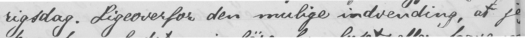rigsdag. Ligeoverfor den mulige indvending, at jeg
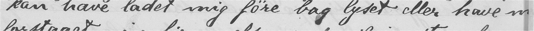kan have ladet mig føre bag lyset eller have mis-
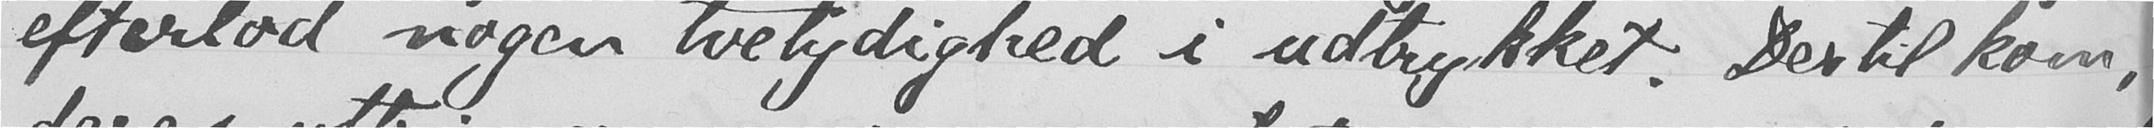efterlod nogen tvetydighed i udtrykket. Dertil kom,
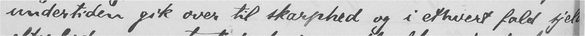undertiden gik over til skarphed og i ethvert fald sjelden
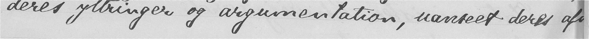deres yttringer og argumentation, uanseet deres af
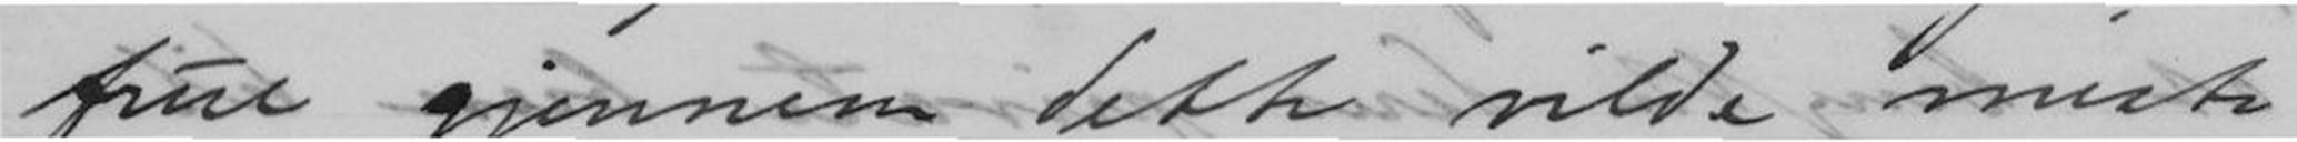deres frue gjennem dette vilde miste
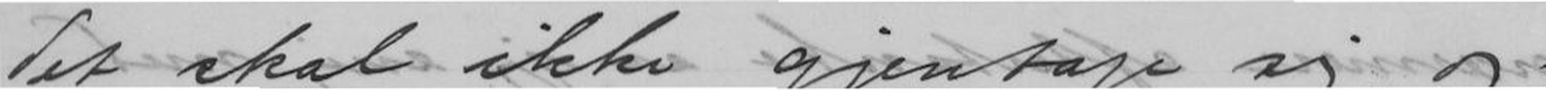det skal ikke gjentage sig, og - jeg
